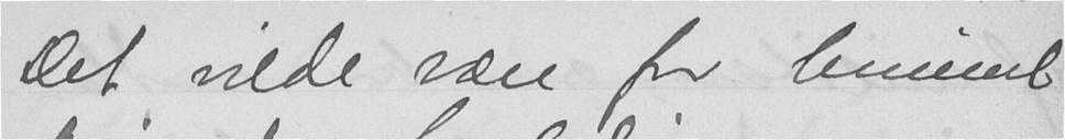Det vilde være for himmel-
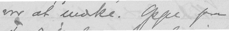var at mærke. Oppe paa
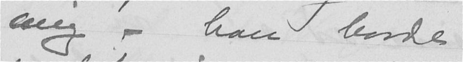mig - han havde
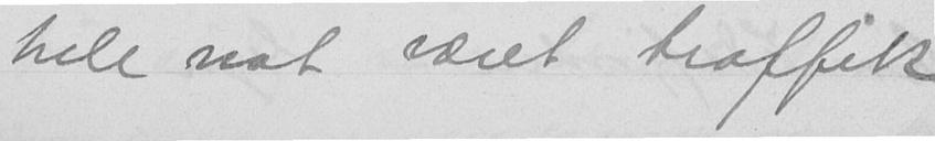hele nat været traffik
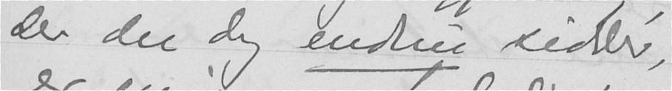der der dog endnu sidder,
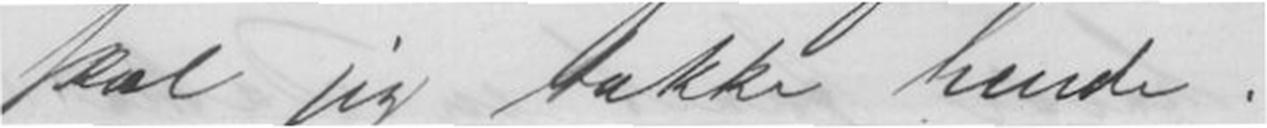skal jeg takke hende!
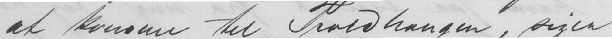at komme til Troldhaugen, siger
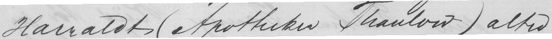Harraldt (Apotheker Thaulow) altid
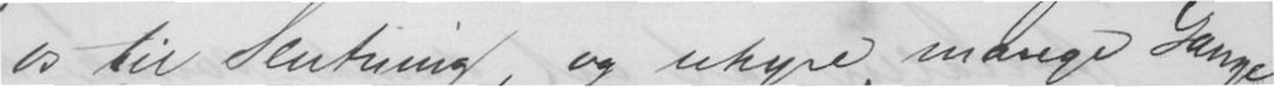os til Sentning, og uhyre mange Gange
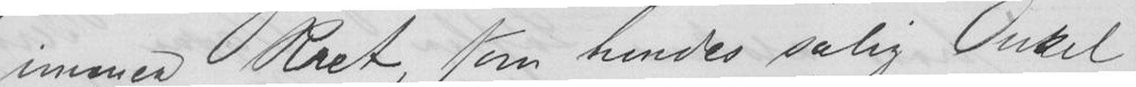immer Reet, som hendes salig Onkel
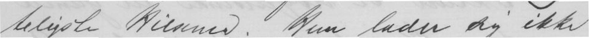teligste Hilsener. Hun lader sig ikke
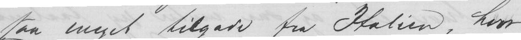saa meget tilgode for Italien, hvor
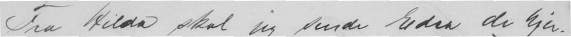Fra Hilda skal jeg sende Eder de hjer-
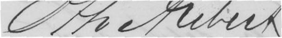Otto Aubert
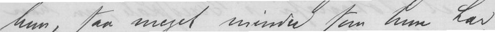hun, saa meget mindre som hun har
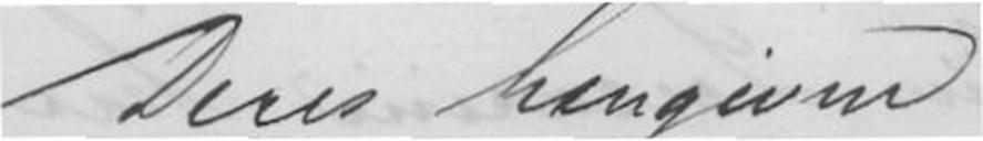Deres hengivne
